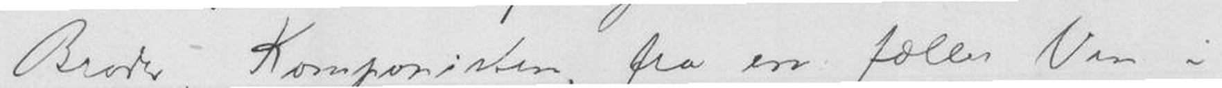Broder, Komponisten, fra en fælles Ven i
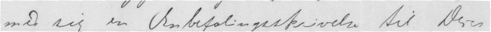med sig en Anbefalingsskrivelse til Deres
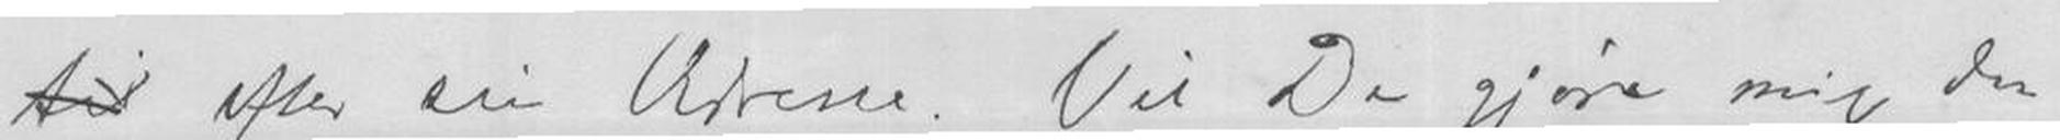til efter sin Adresse. Vil De gjøre mig den
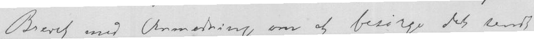Brevet med Anmodning om at besørge det sendt
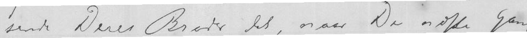sende Deres Broder det, naar De gang
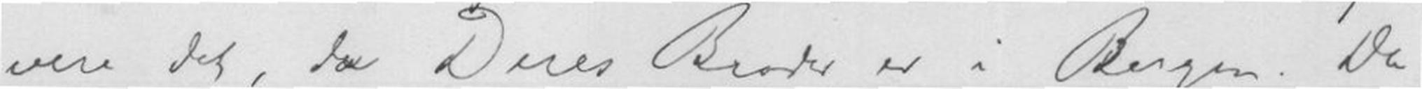vere det, da Deres Broder er i Bergen. Da
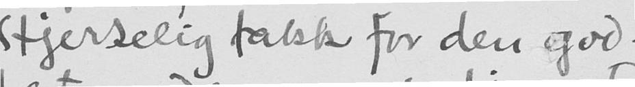Hjertelig takk for den god-
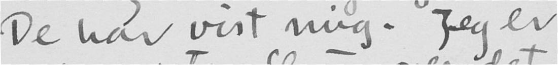De har vist mig. Jeg er
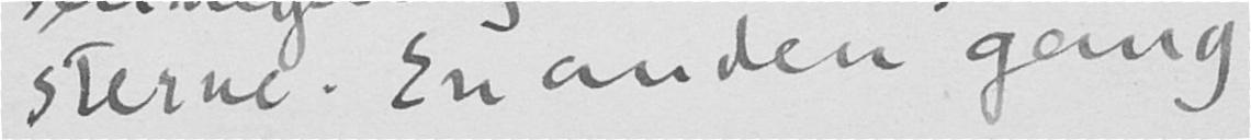sterne. En anden gang
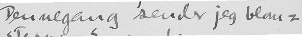Dennegang sender jeg blom-
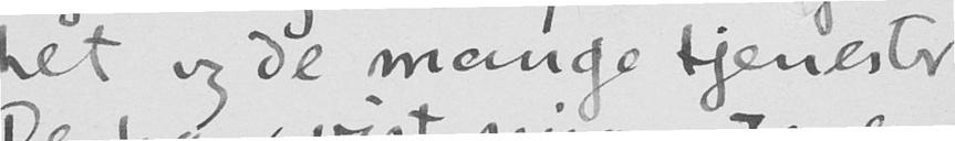het og de mange tjenester
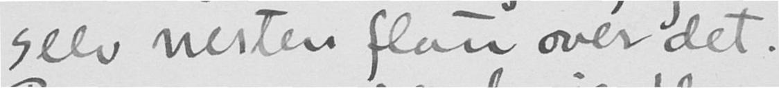selv nesten flau over det.
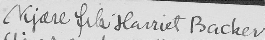Kjære frk Harriet Backer
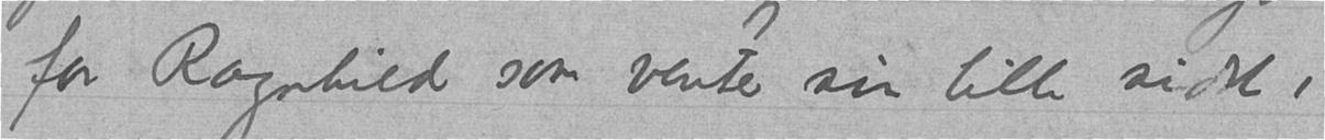for Ragnhild som venter sin lille sidst i
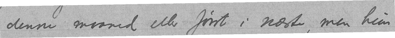denne maaned eller først i næste, men hun
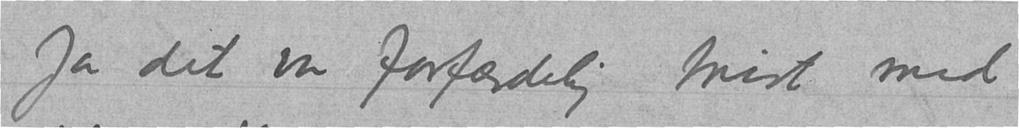Ja det var forfærdelig trist med
